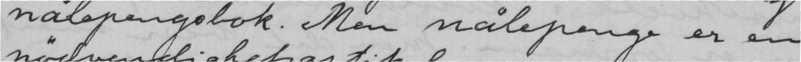nålepengebok. Men nålepenge er en
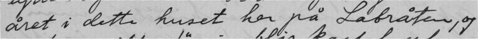året i dette huset her på Labråten, og
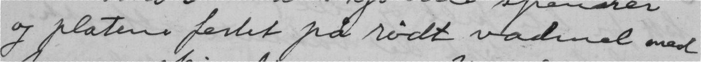og platene festet på rødt vadmel med
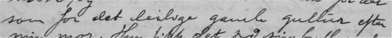som for det deilige gamle gullur efter
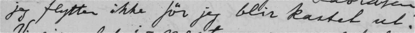Jeg flytter ikke før jeg blir kastet ut.
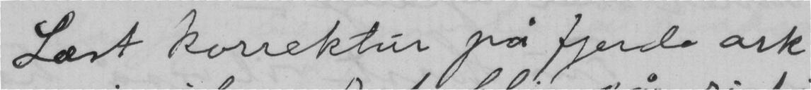Læst korrektur på fjerde ark
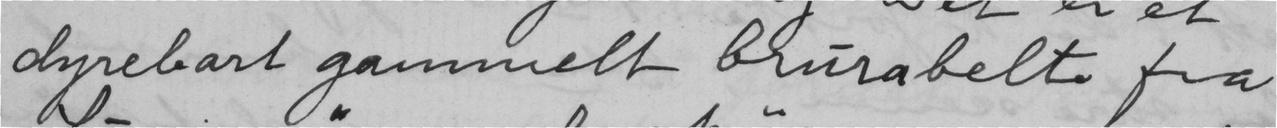dyrebart gammelt brurabelte fra
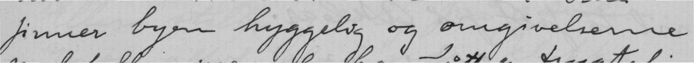finner byen hyggelig og omgivelserne
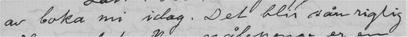av boka mi idag. Det blir sån rigtig
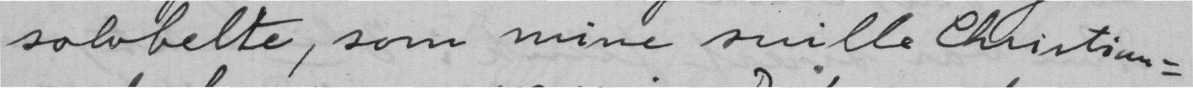sølvbelte, som mine snille Christian-
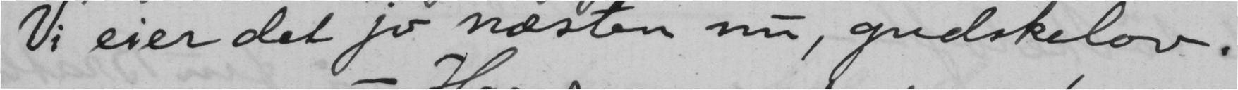Vi eier det jo næsten nu, gudskelov.
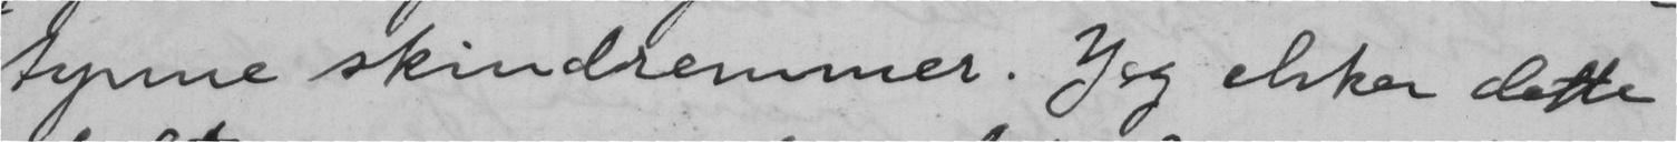tynne skindremmer. Jeg elsker dette
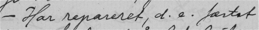Har repareret, d. e. fæstet
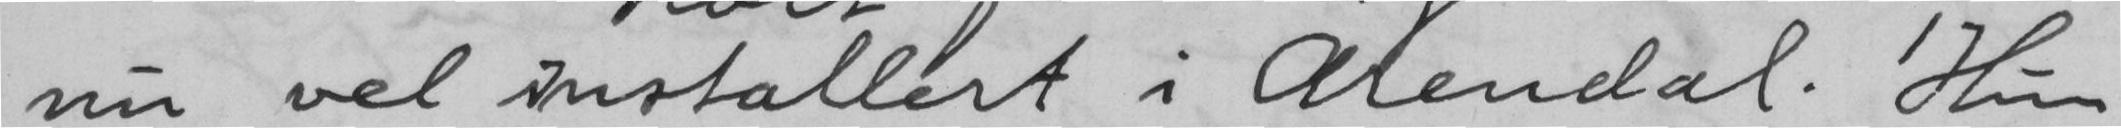nu vel installert i Arendal. Hun
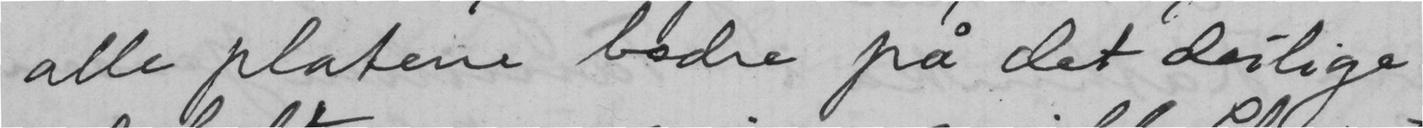alle platene bedre på det deilige
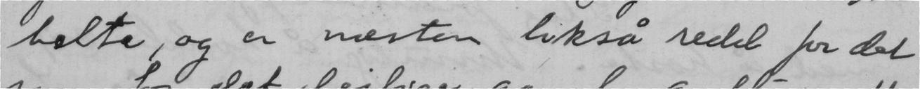belte, og er næsten likså redd for det
